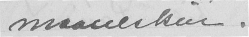maaneskin.
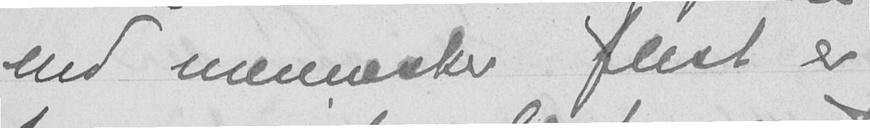end mennesker flest er
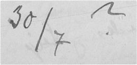30/7 ?
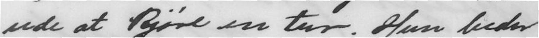ude at kjøre en tur. Hun beder
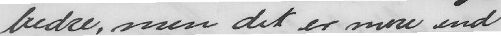bedre, men det er mere end
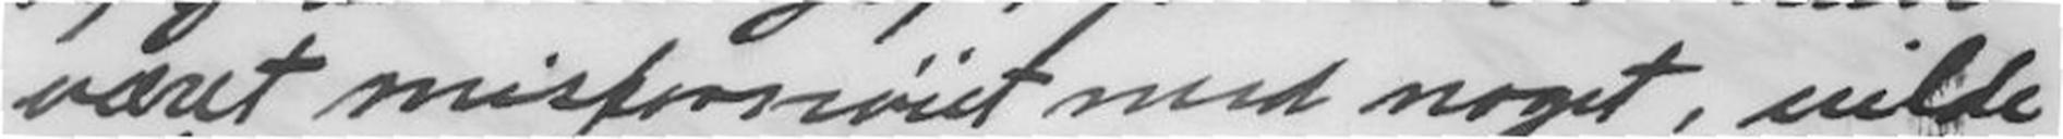været misfornøiet med noget, vilde
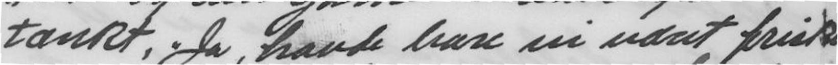tænkt, Ja, havde bare vi vært friske
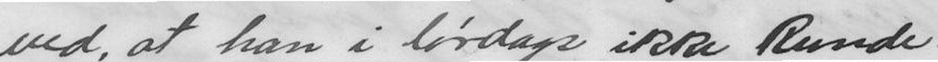ved, at han i lørdags ikke kunde
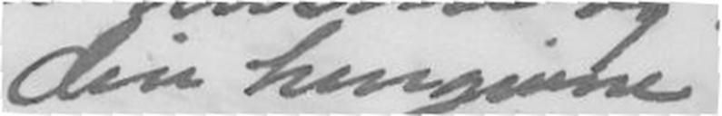din hengivne
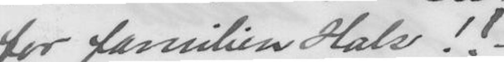for familien Hals!!
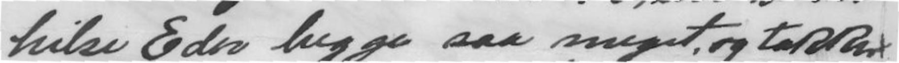hilse Eder begge saa meget, og takker
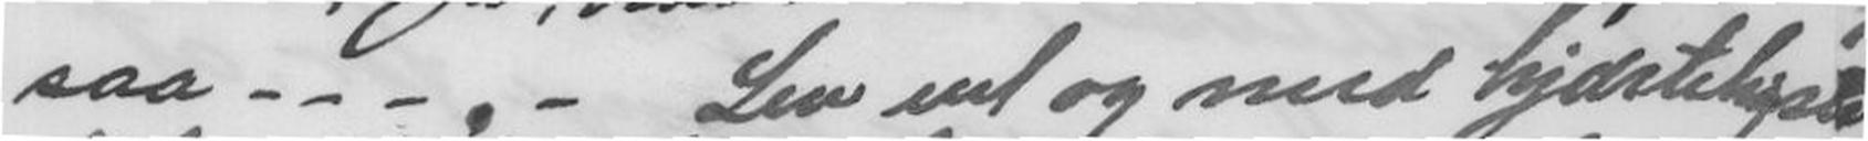saa---.- Lev vel og med hjærteligste
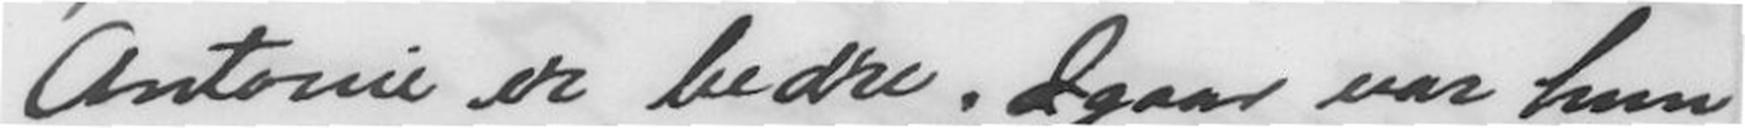Antonie er bedre. Igaar var hun
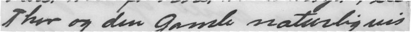Thor og den Gamle naturligvis
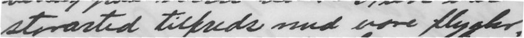storartet tilfreds med vore flygler,
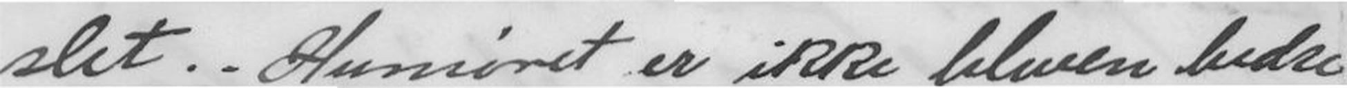slet. - Humøret er ikke bleven bedre
